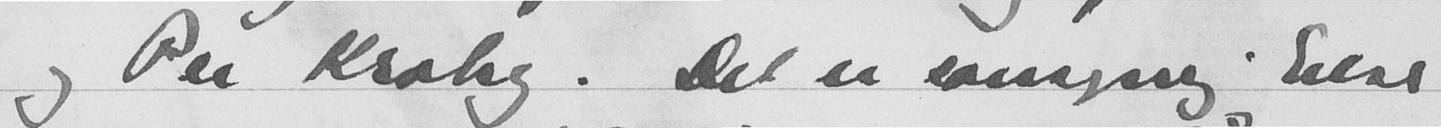og Per Krohg. Det er saengnig Else
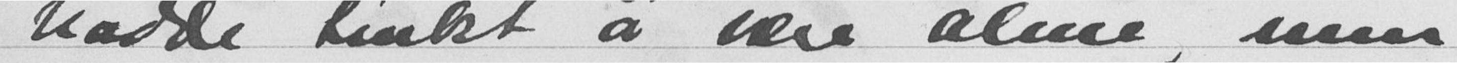hadde tenkt å være alene, men
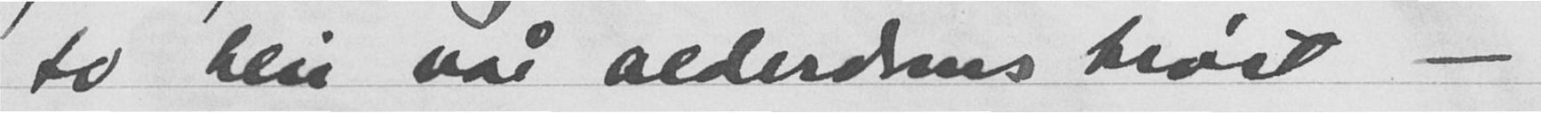to blir vår alderdoms trøst -
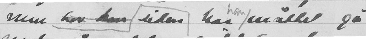men  siden har han måttet gå
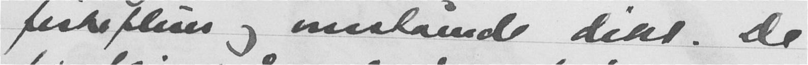fiskefluer og enestående dikt. De
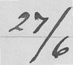27/6
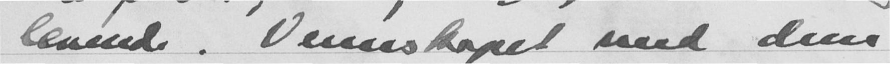levende. Vennskapet med dem
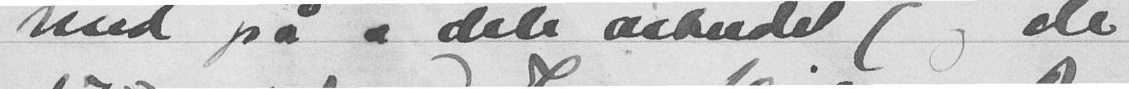med på å del arbeide (og de
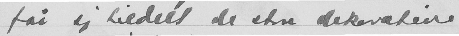får, sig tildelt de store dekorative
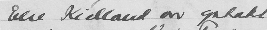Else Kielland var optatt
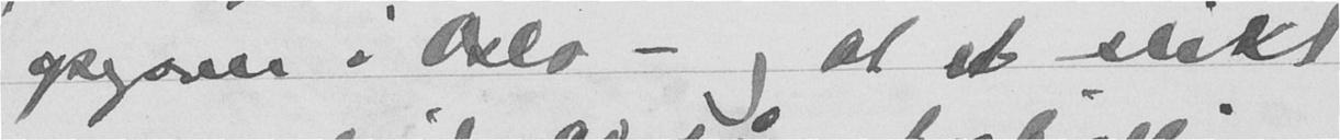opgaver i Oslo - og at et slikt
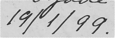19/1/99.
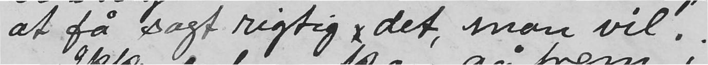at få sagt rigtig det, man vil.
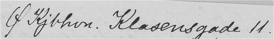Ø Kjbhvn Klasensgade 11.
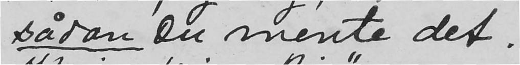såden Du mente det.
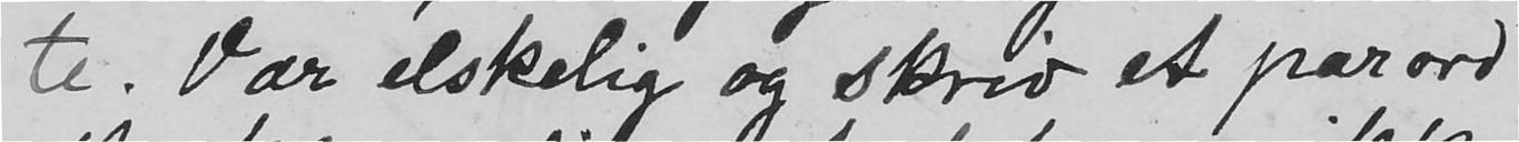te. Vær elskelig og skriv et par ord
